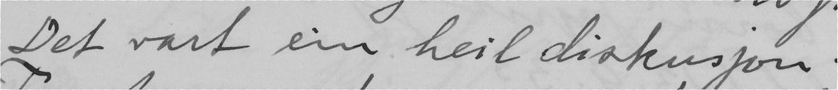Det vart ei heil diskusjon.
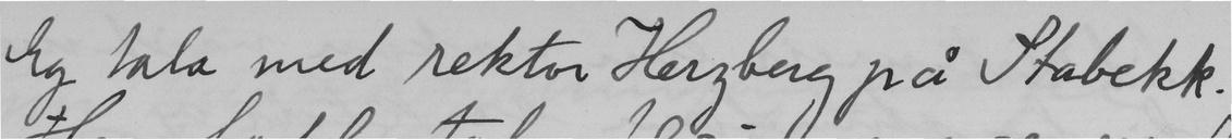Eg tala med rektor Herzberg på Stabekk.
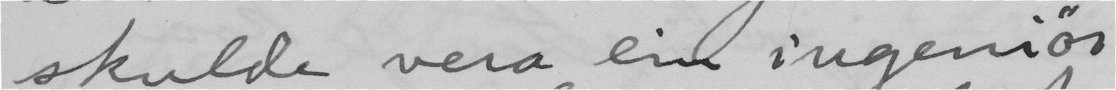skulde vera en ingeniör
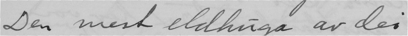Den mest eldhuga av dei
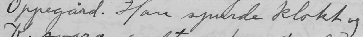Oppegård. Han spurde klokt og
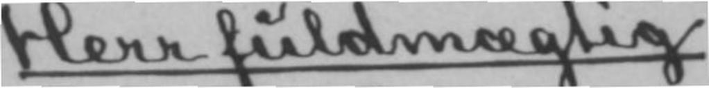Herr fuldmægtig
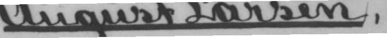August Larsen,
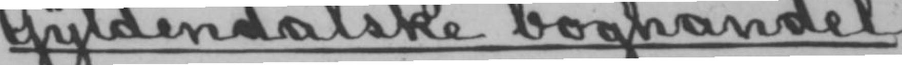Gyldendalske boghandel,
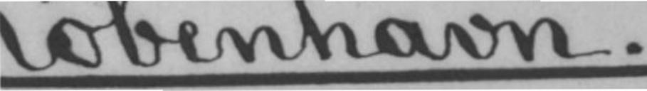København.
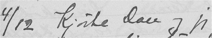4/12 Kjørte Don og jeg
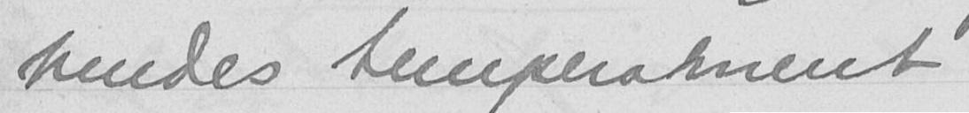hendes temperament
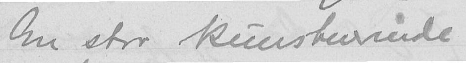En stor kunstnerinde
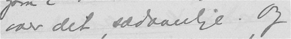over det sædvanlige. Og
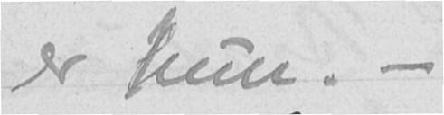er hun. -
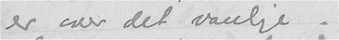er over det vanlige.
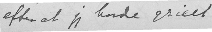efter at jeg havde greiet
